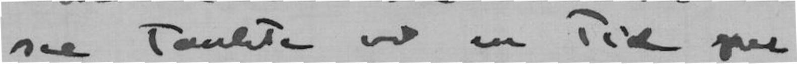saa Tænkte vi en Tid paa
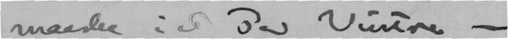- maaske i Paa Vintre -
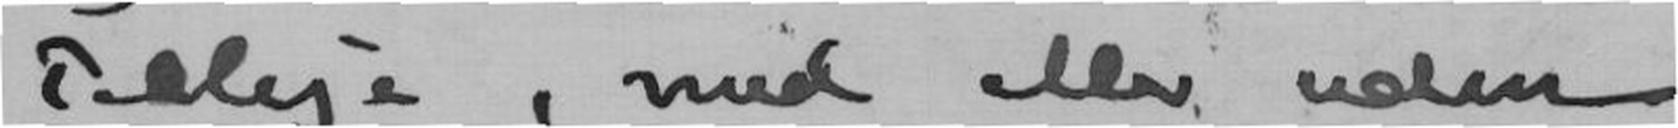Tilleje, med eller uden
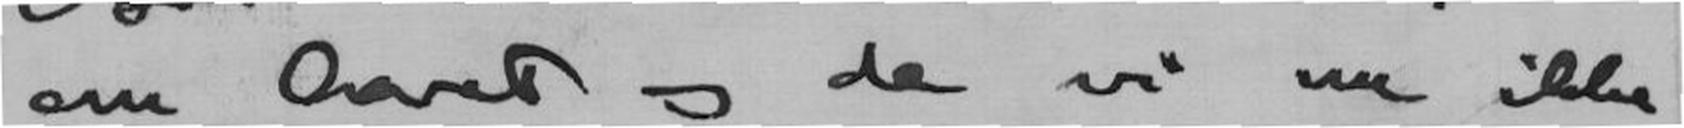om Aaret og da vi nu ikke
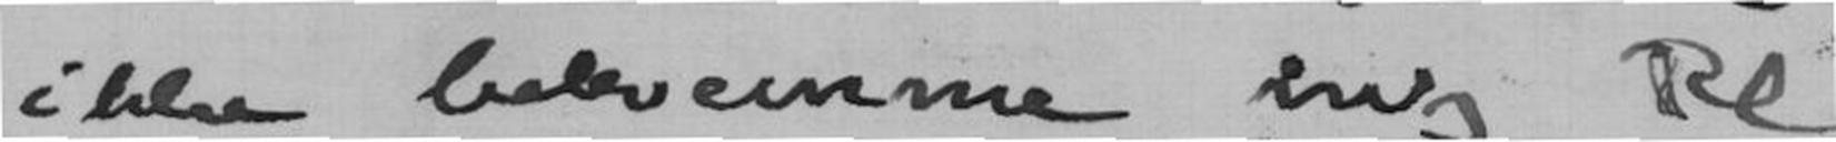ikke bestemme mig til
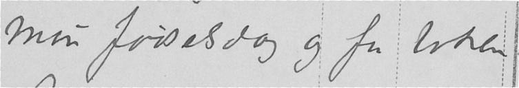min fødselsdag og for boken.
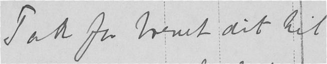Tak for brevet dit til
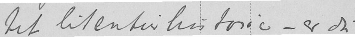tet literaturhistorie - er du
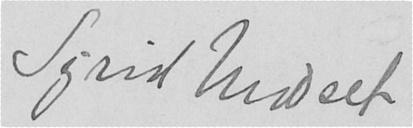Sigrid Undset
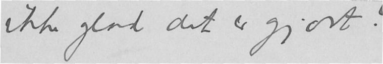ikke glad det er gjort?
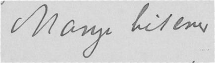Mange hilsener
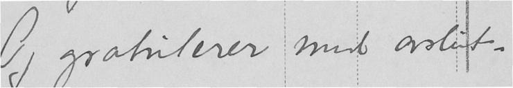Og gratulerer med avslut-
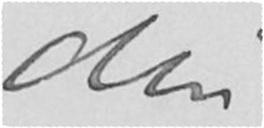din
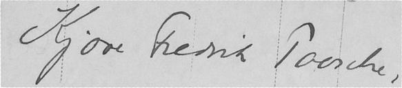Kjære Fredrik Paasche,
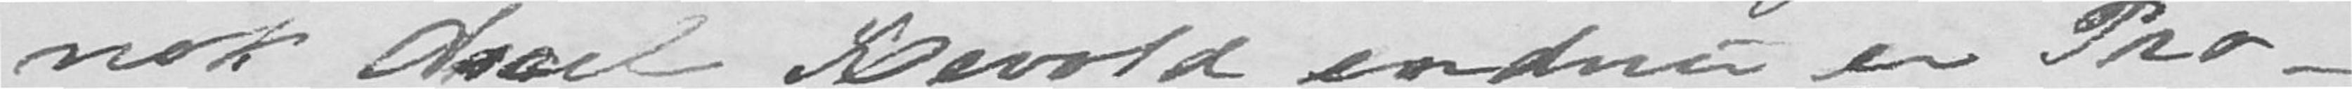nok Axel Revold endnu en Pro-
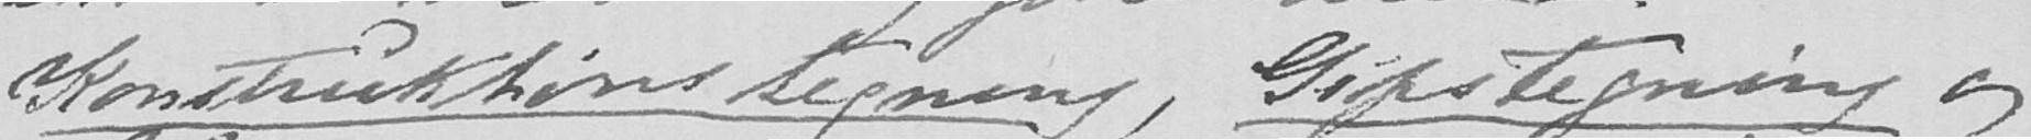Konstruktionstegning, Gipstegning og
